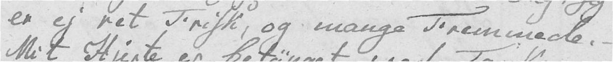er ej ret Frisk, og mange Fremmede. –
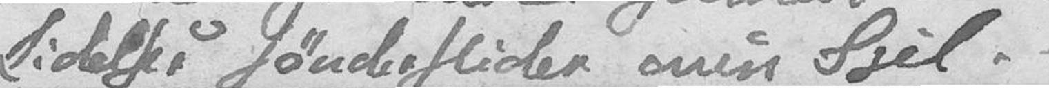Lidelser sønderslider min Sjel. –
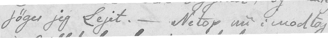søger jeg Lejet. – Netop nu imodtog
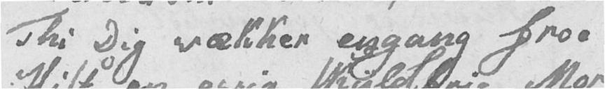Thi Dig vækker engang froe
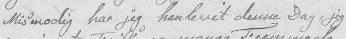Mismodig har jeg henlevet denne Dag, jeg
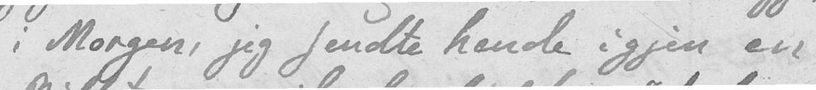i Morgen, jeg sendte hende igjen en
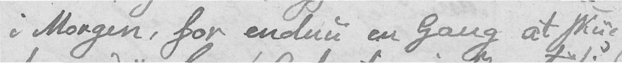i Morgen, for endnu en Gang at skue
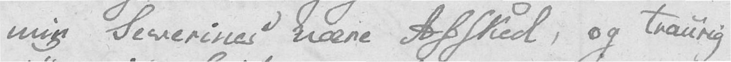min Severines nære Afsked, og traurig
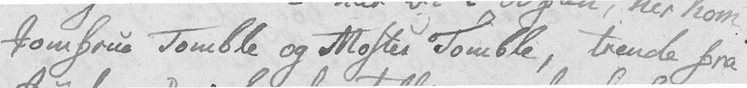Jomfrue Tomble og Moster Tomble, tvende fra
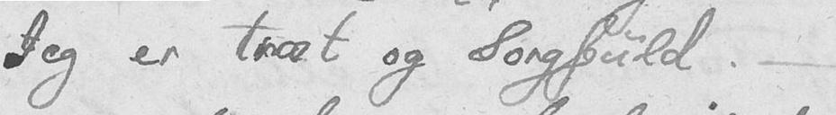Jeg er træt og Sorgfuld. –
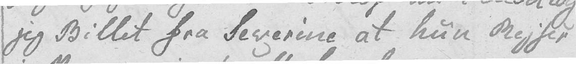jeg Billet fra Severine at hun Rejser
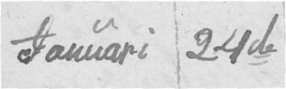Januari 24de
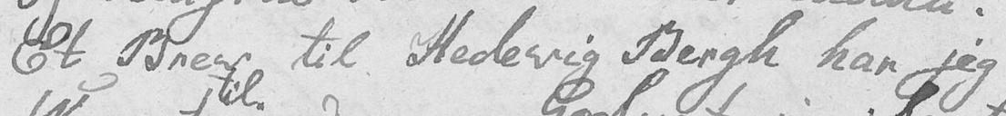Et Brev til Hedevig Bergh har jeg
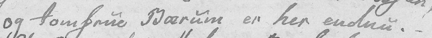og Jomfrue Bærum er her endnu. –
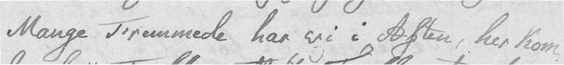Mange Frremmede har vi i Aften, her kom
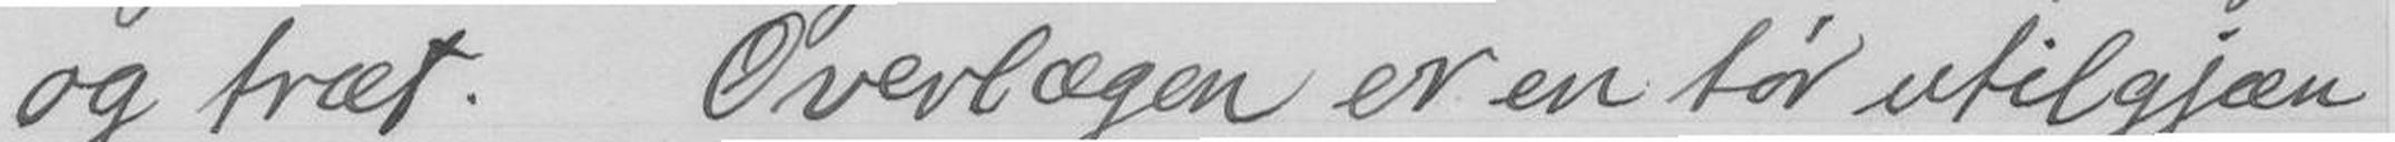og træt. Overlægen er en tør utilgjæn-
